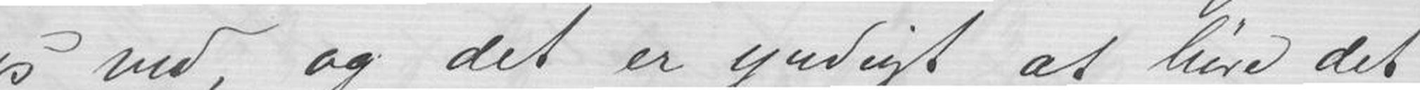os ved, og det er yndigt at høre det
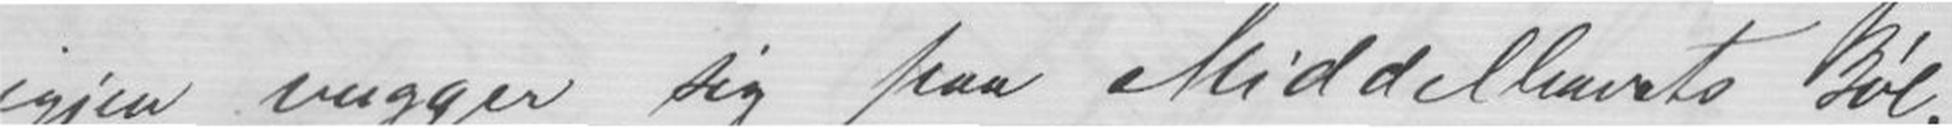igjen vugger sig paa Middelhavets Bøl-
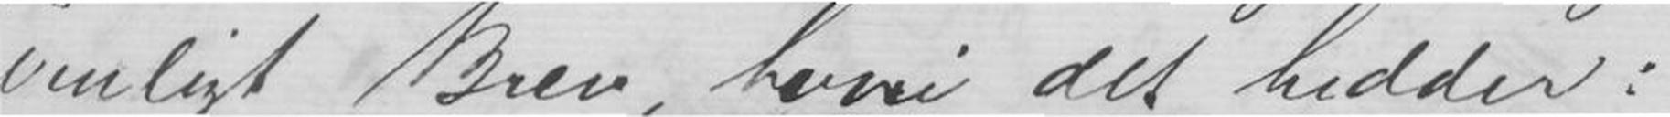venligt Brev, hvori det hedder:
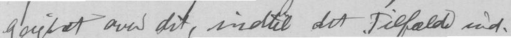geistret over det, indtil det Tilfælde ind-
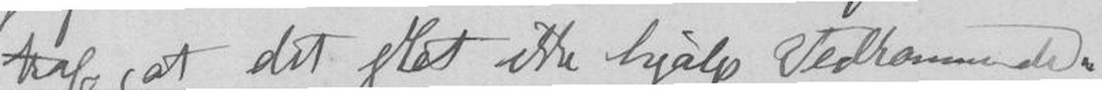traf, at det slet ikke hjalp Vedkommende.
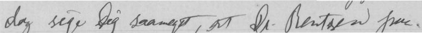dog sige Dig saameget, at Dr. Berntsen paa
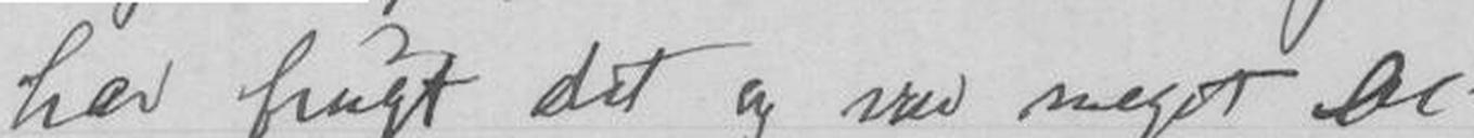har brugt det og var meget be-
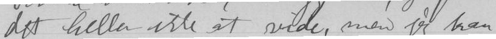det heller ikke at sidde, men jeg kan
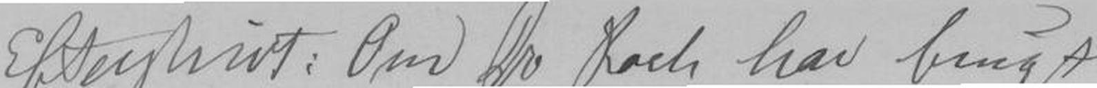Efterskrivt: Om Dr Roch har brugt
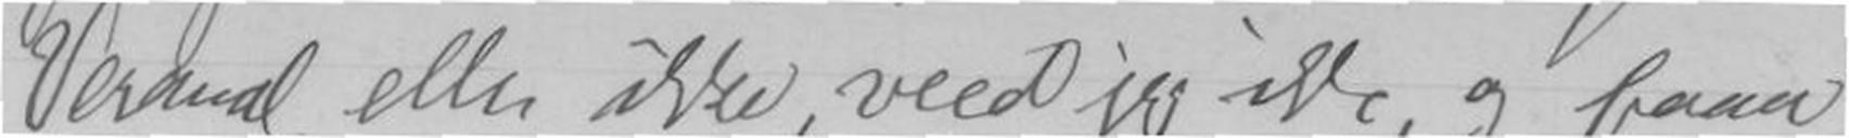Veranel?? eller ikke, veed jeg ikke, og faaer
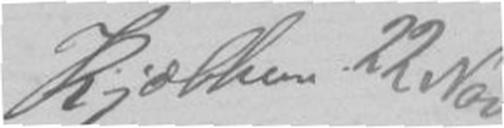Kjøbenhavn 22 nov.
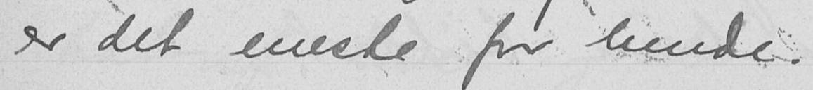er det eneste for hende.
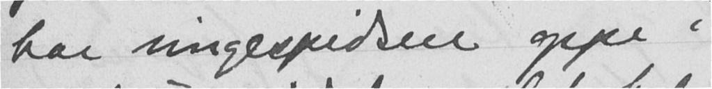har vingespidsen oppe i
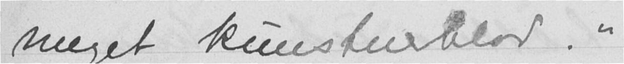meget kunstnerblod."
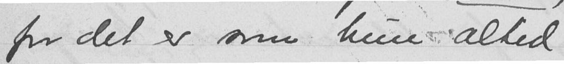for det er som hun altid
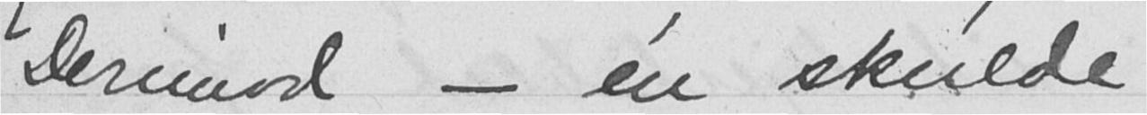Derimod - en skulde
